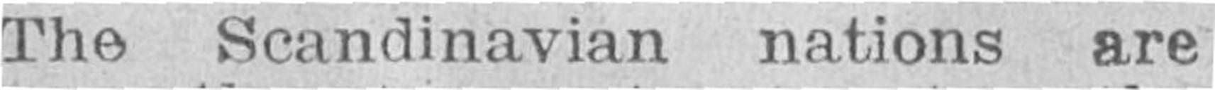The Scandinavian nations are
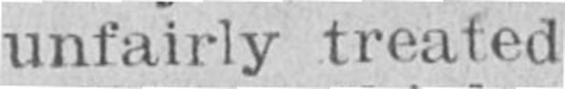unfairly treated
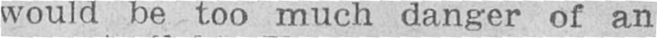would be too much danger of an
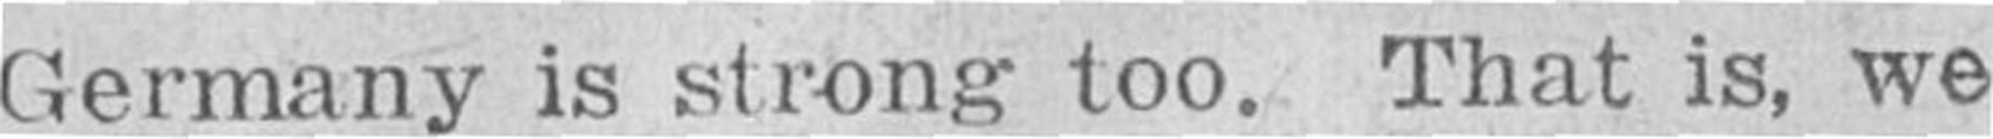Germany is strong too. That is, we
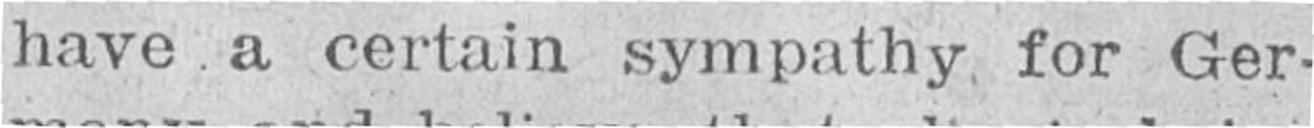have a certain sympathy for Ger-
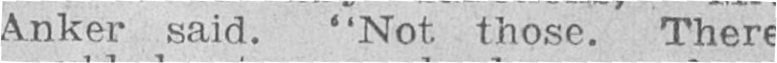Anker said. "Not those. There
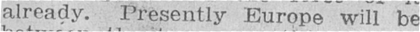already. Presently Europe will be
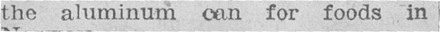the aluminum can for foods in
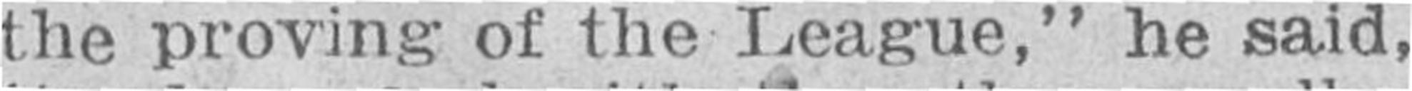the proving of the League," he said,
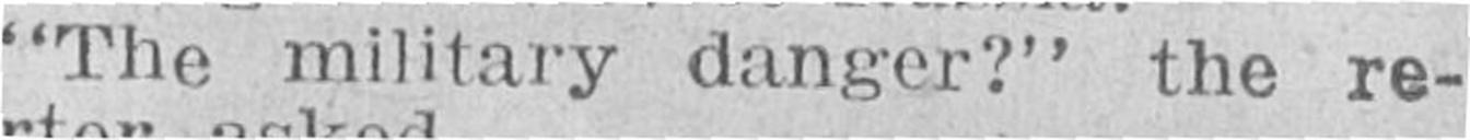"The military danger?" the re-
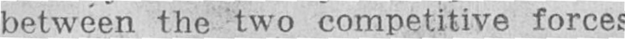between the two competitive forces
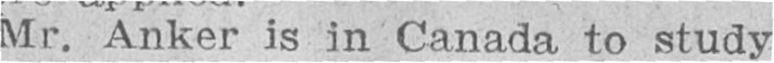Mr. Anker is in Canada to study
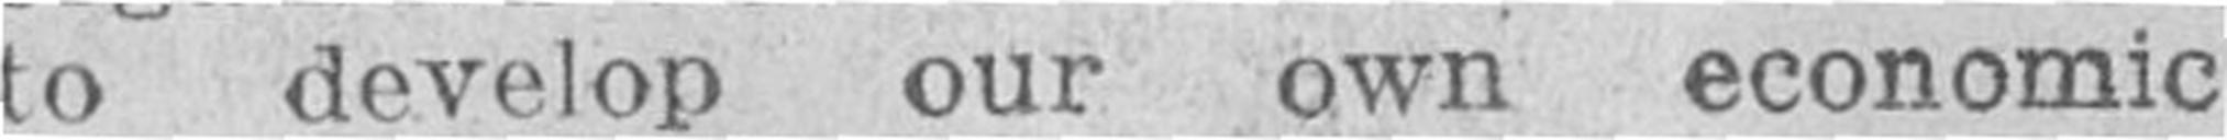to develop our own economic
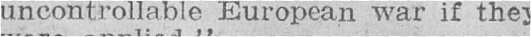uncontrollable European war if they
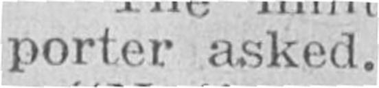porter asked.
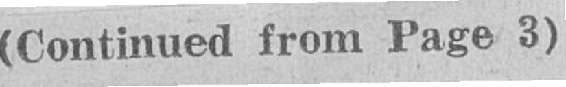(Continued from Page 3)
